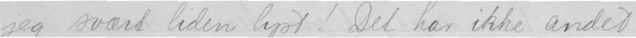jeg svært liden lyst! Det har ikke andet
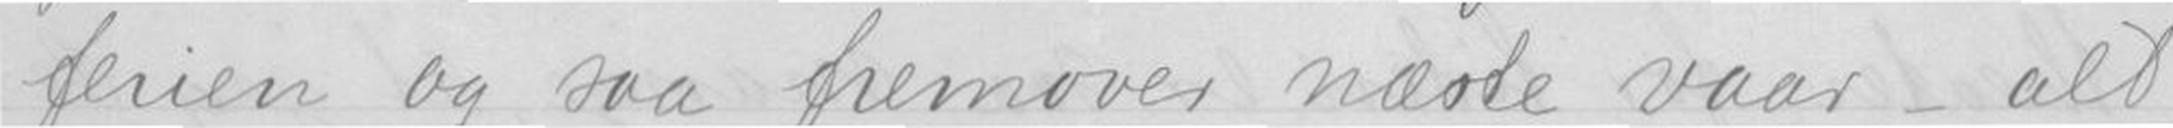ferien og saa fremover næste vaar - alt
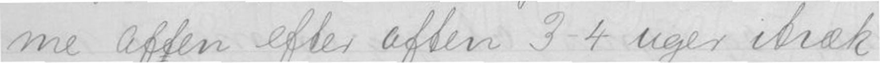me aften efter aften 3-4 uger itræk.
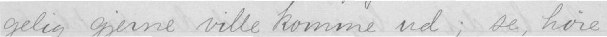gelig gjerne ville komme ud; se, høre
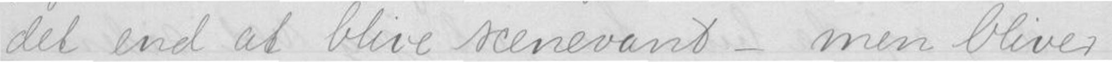det end at blive scenevant _ men bliver
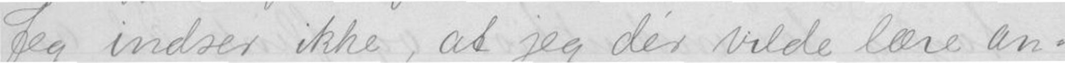Jeg indser ikke, at jeg der vilde lære an-
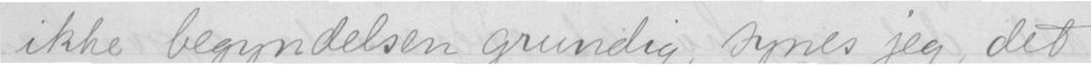ikke begyndelsen grundig, synes jeg, det
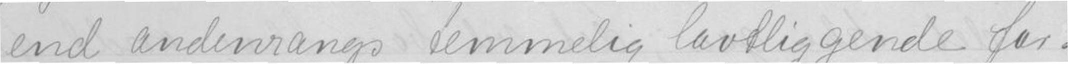end andenrangs temmelig lavtliggende far-
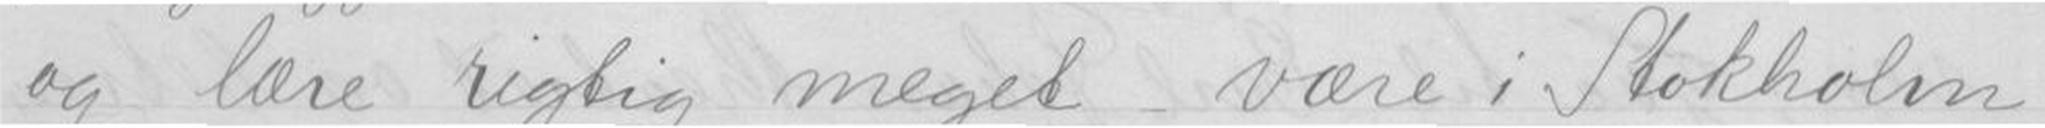og lære rigtig meget - være i Stokholm
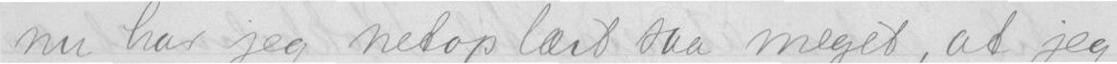nu har jeg netop læst saa meget, at jeg
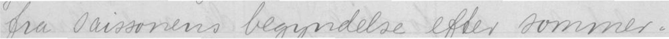fra saissonens begyndelse efter sommer-
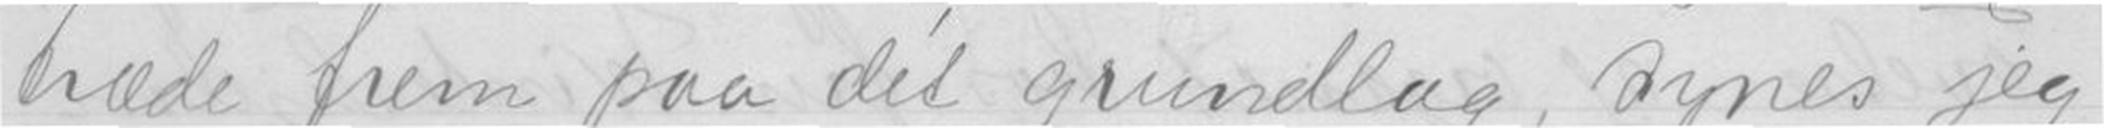træde frem paa dèt grundlag, synes jeg
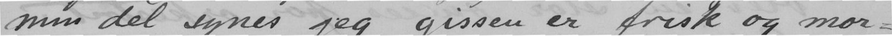min del synes jeg gissen er frisk og mor-
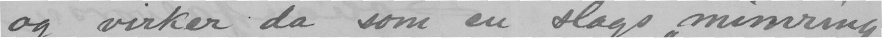og virker da som en slags mimring.
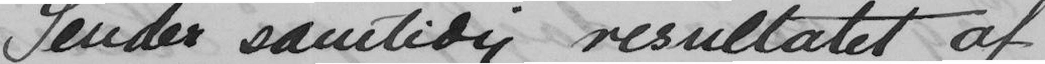Sender samtidig resultatet af
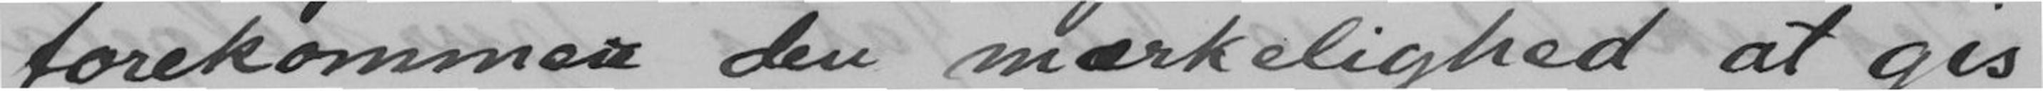forekommer den mærkelighed at gis
